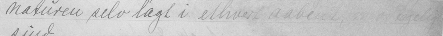naturen selv lagt i et hvert aabent, modtageligt
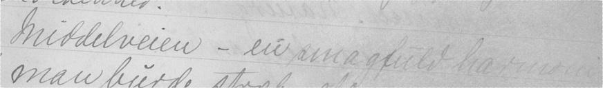Middelveien - en smagfuld harmoni
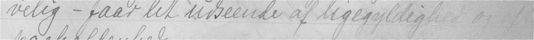velig - faar let udseende af ligegyldighed og ofte
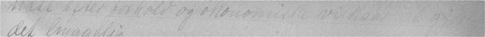næst efter forhold og økonomiserte vilkaar at gjøre
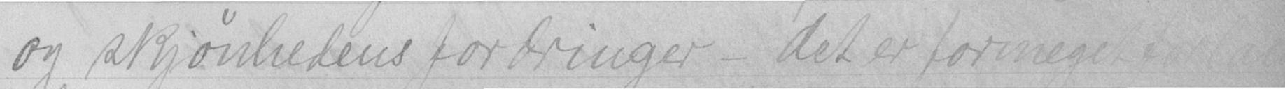og skjønhedens fordringer - det er formeget
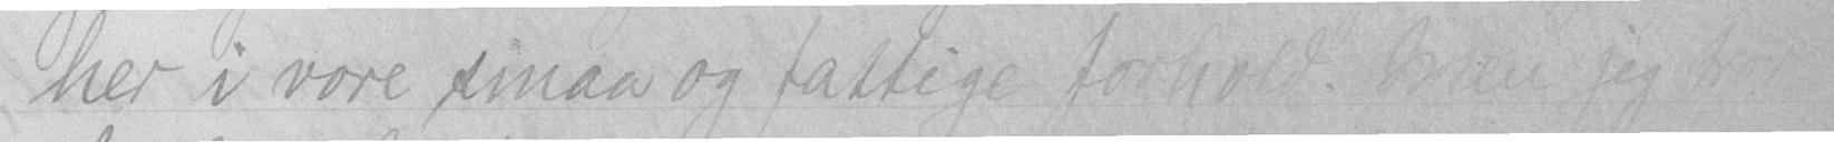her i vore smaa og fattige forhold. Men jeg tror
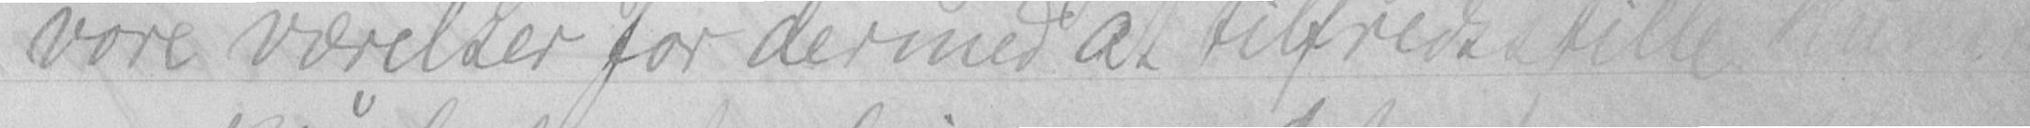vore værelser for dermed at tilfredsstille kunstens
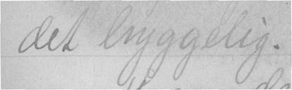det hyggelig.
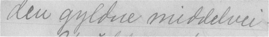den gyldne middelvei!
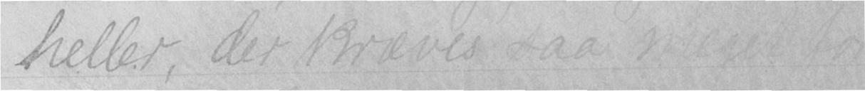heller, der kræves saa meget for
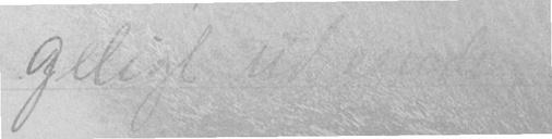geligt udseende,
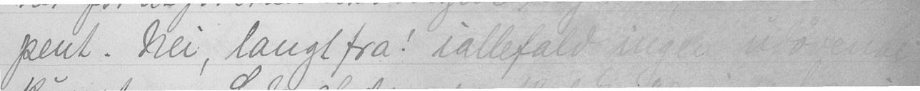pent. Nei, langt fra! iallefald ingen udøvende
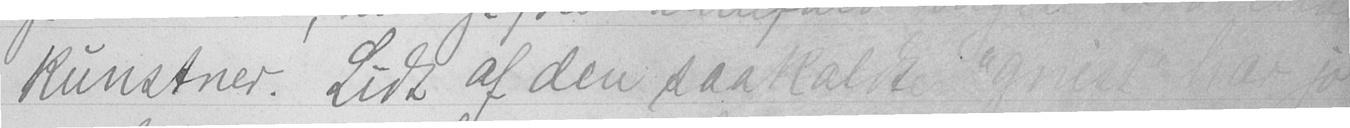kunstner. Lidt af den saakaldte "gnist" har jo
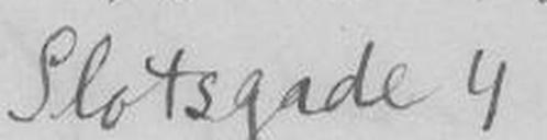Slotsgade 4
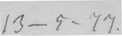13- 5-77.
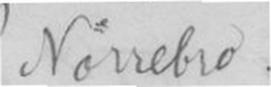Nørrebro.
